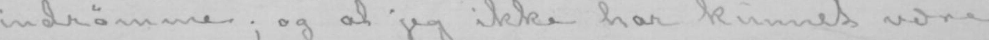indrømme; og at jeg ikke har kunnet være
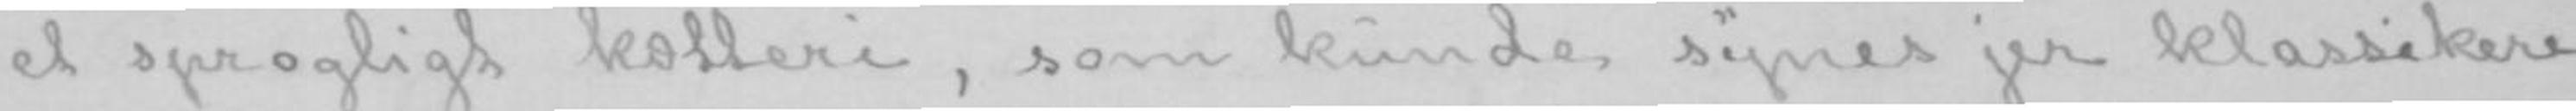et sprogligt kætteri, som kunde synes jer klassikere
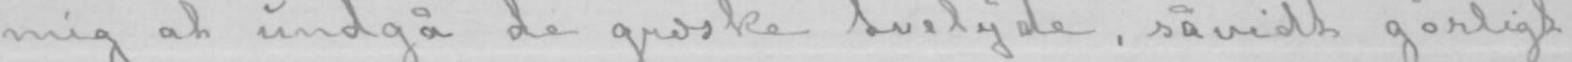mig at undgå de græske tvelyde, såvidt gørligt.
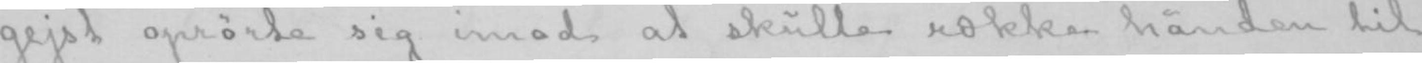gejst oprørte sig imod at skulle række hånden til
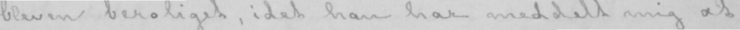bleven beroliget, idet han har meddelt mig at
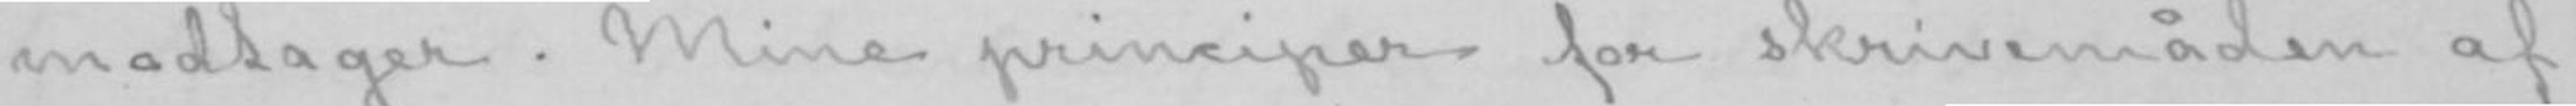modtager. Mine principer for skrivemåden af
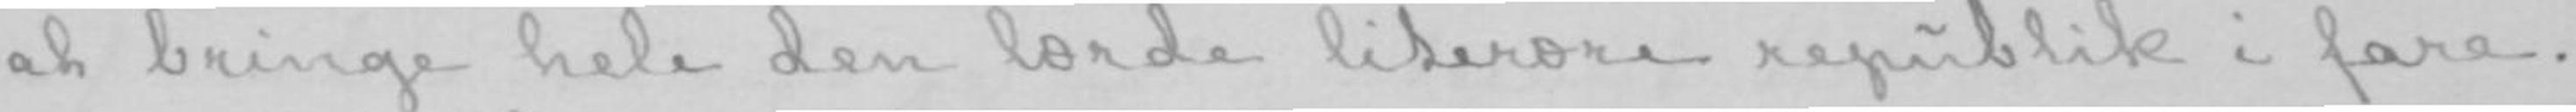at bringe hele den lærde literære republik i fare.
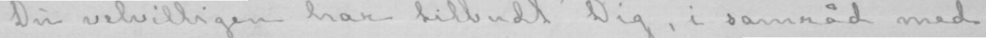Du velvilligen har tilbudt Dig, i samråd med
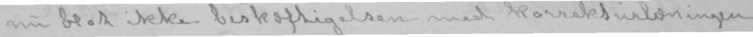nu blot ikke beskæftigelsen med korrekturlæsningen
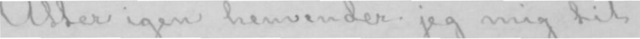Atter igen henvender jeg mig til Dig
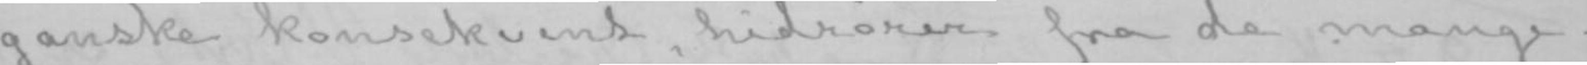ganske konsekvent, hidrører fra de mange-
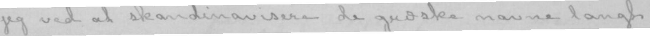jeg ved at skandinavisere de græske navne langt
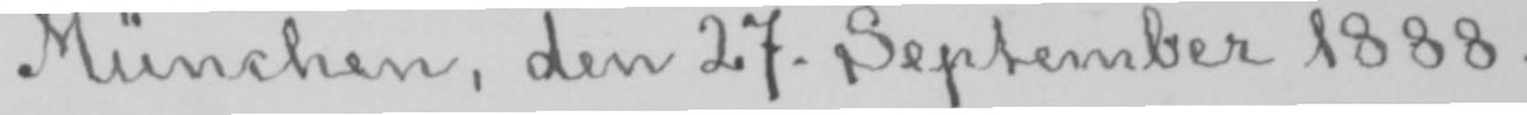München, den 27. September 1888.
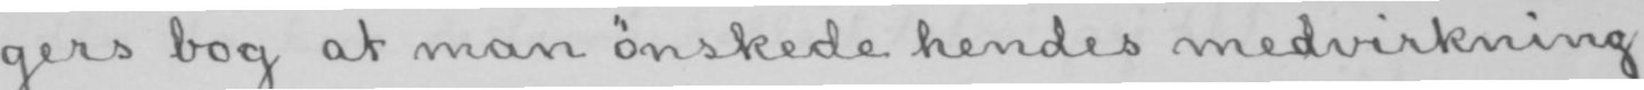gers bog at man ønskede hendes medvirkning.
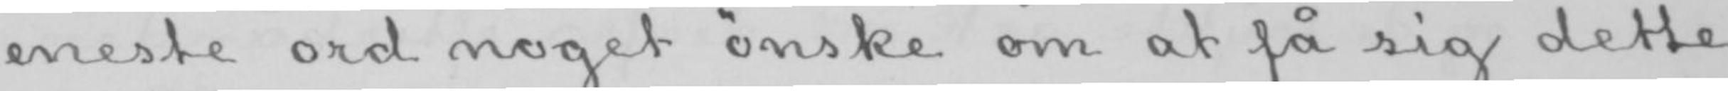eneste ord noget ønske om at få sig dette
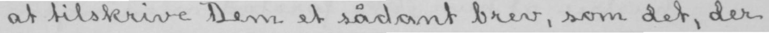at tilskrive Dem et sådant brev, som det, der
